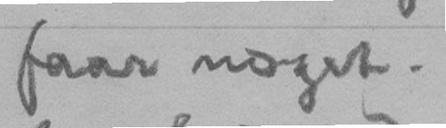faar noget.
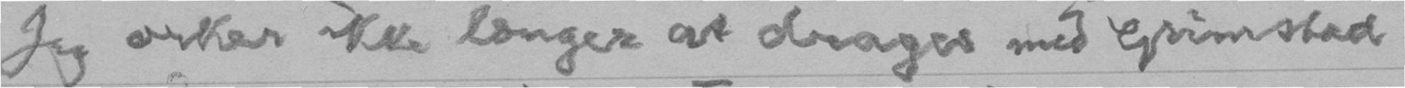Jeg orker ikke længer at drages med Grimstad
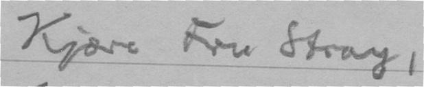Kjære Fru Stray,
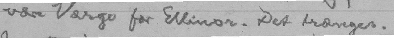være Værge for Ellinor. Det trænges.
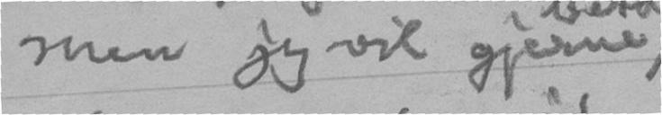men jeg vil gjerne
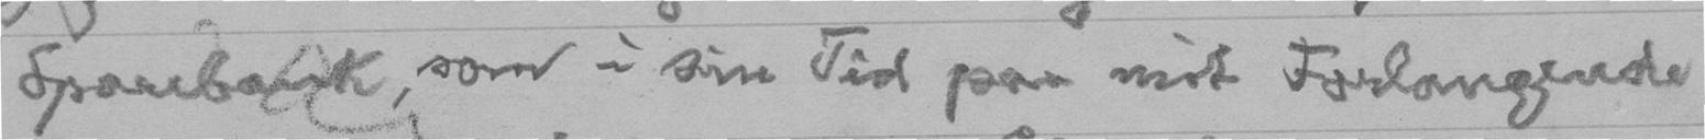Sparebank, som i sin Tid paa mit Forlangende
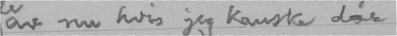av nu hvis jeg kanske dør
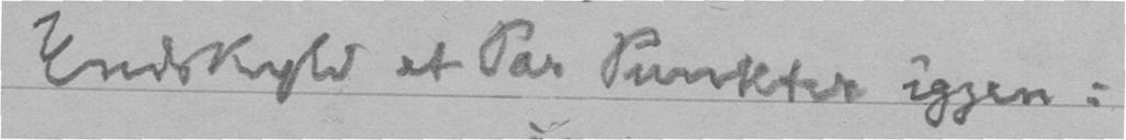Undskyld et Par Punkter igjen:
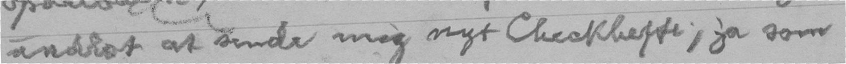undlot at sende mig nyt Checkhefte, ja som
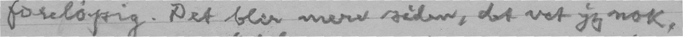foreløpig. Det blir mere siden, det vet jeg nok,
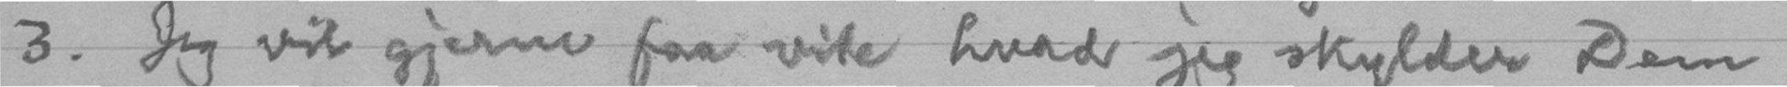3. Jeg vil gjerne faa vite hvad jeg skylder Dem
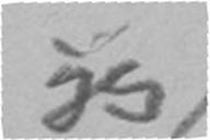jeg
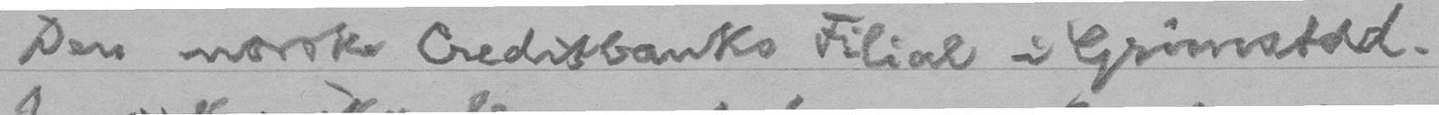Den norske Creditbanks Filial i Grimstad.
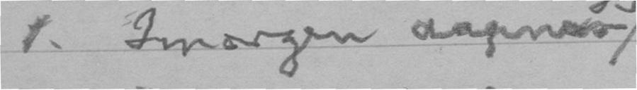1. Imorgen aapner
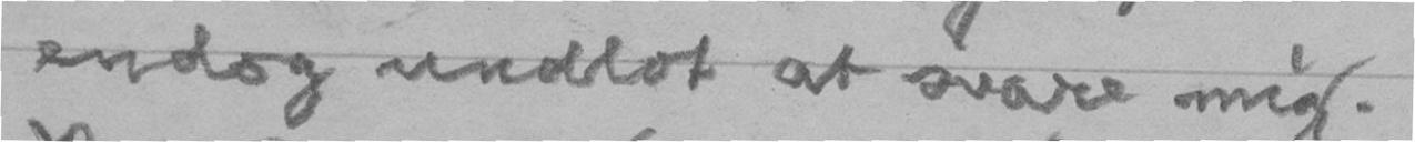endog undlot at svare mig.
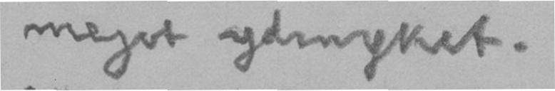meget ydmyket.
